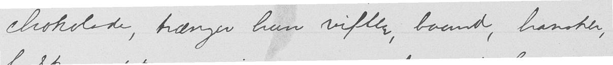chokolade, trænger hun vifter, baand, hansker,
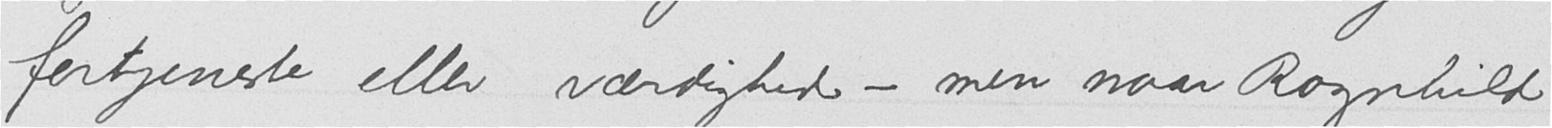fortjeneste eller værdighed - men naar Ragnhild
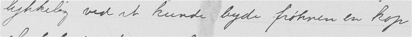lykkelig ved at kunde byde frøknen en kop
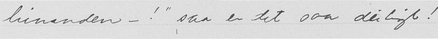hinanden - !" saa er det saa deiligt!
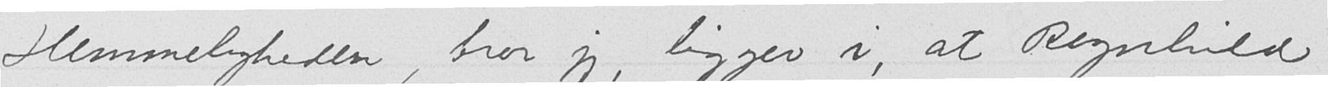Hemmeligheden, tror jeg, ligger i, at Ragnhild
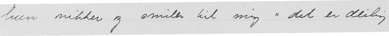hun nikker og smiler til mig "det er deilig
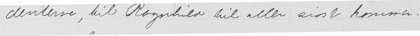denterne, til Ragnhild til aller sist kommer:
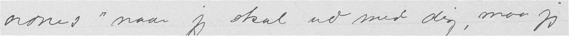ordnes "naar jeg skal ud med dig, maa jeg
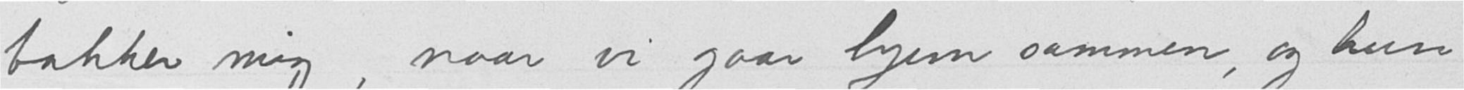takker mig, naar vi gaar hjem sammen, og hun
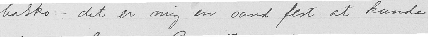balsko - det er mig en sand fest at kunde
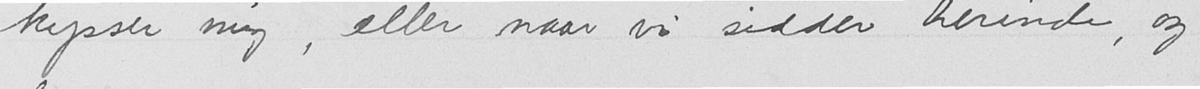kysser mig, eller naar vi sidder herinde, og
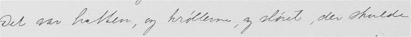Det var hatten, og krøllerne, og sløret, der skulde
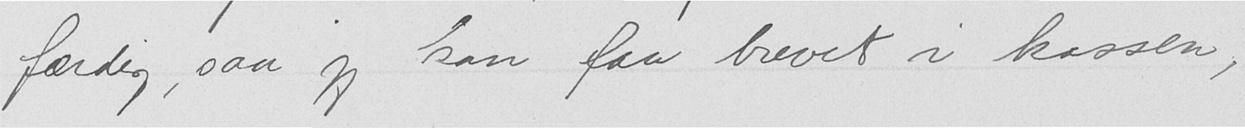færdig, saa jeg kan faa brevet i kassen,
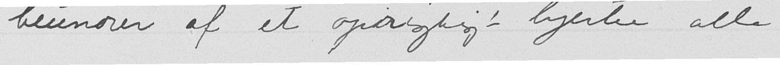beundrer af et oprigtig hjerte alle
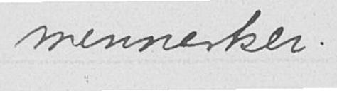mennesker.
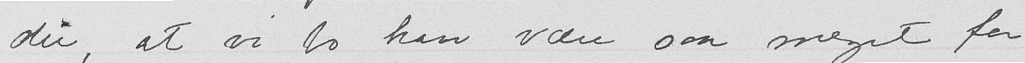du, at vi to kan være saa meget for
